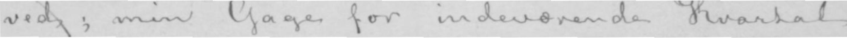ved; min Gage for indeværende Kvartal
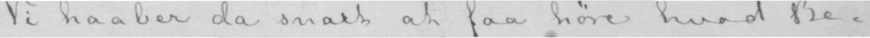Vi haaber da snart at faa høre hvad Be-
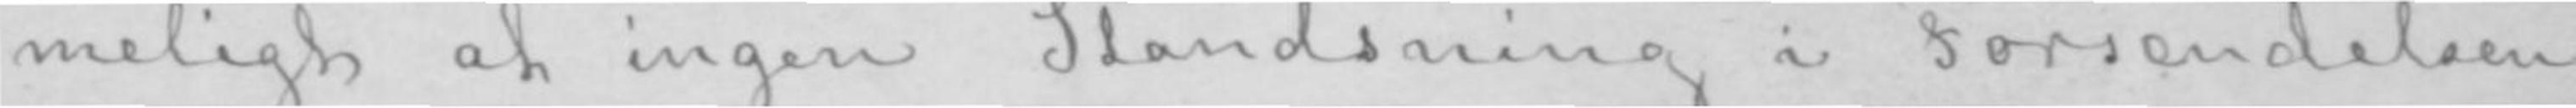meligt at ingen Standsning i Forsendelsen
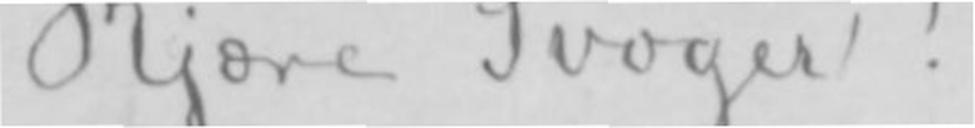Kjære Svoger!
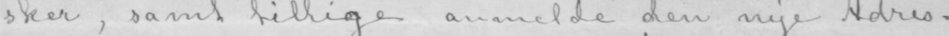sker, samt tillige anmelde den nye Adres-
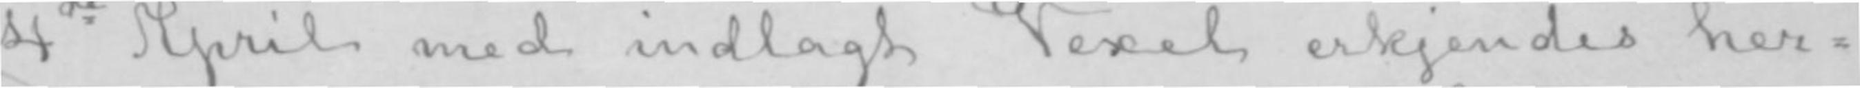4de April med indlagt Vexel erkjendes her-
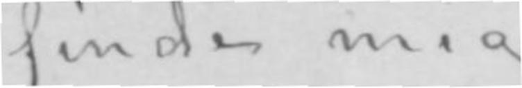finde mig.
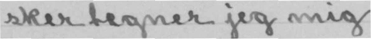sker tegner jeg mig
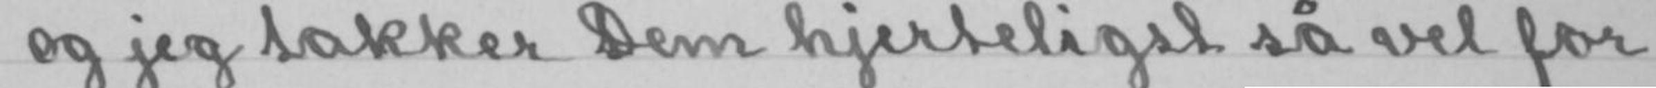og jeg takker Dem hjerteligst så vel for
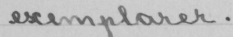exemplarer.
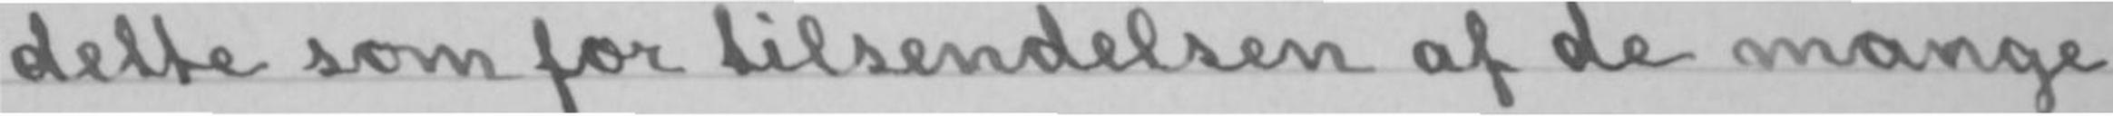dette som for tilsendelsen af de mange
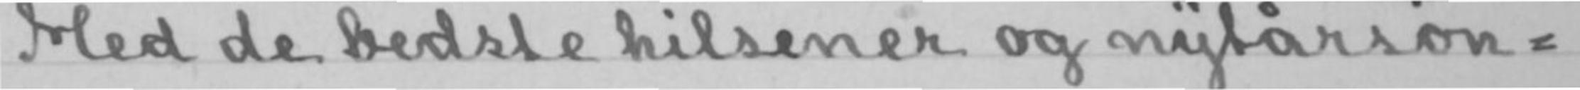Med de bedste hilsener og nytårsøn-
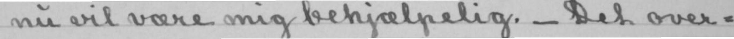nu vil være mig behjælpelig.- Det over-
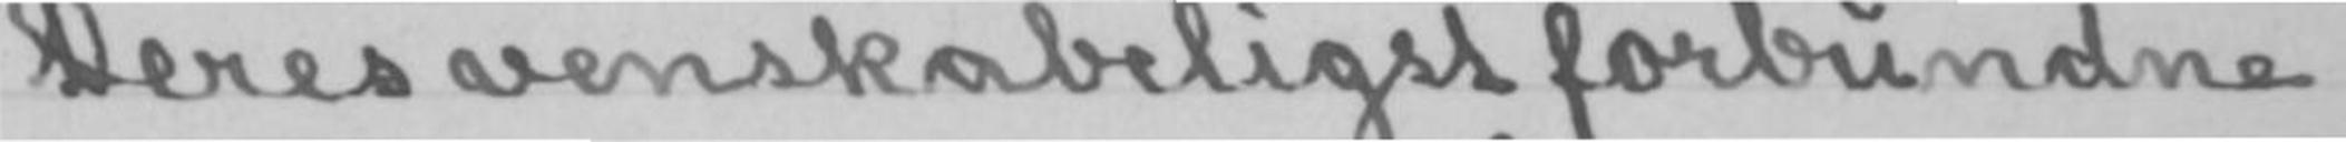Deres venskabeligst forbundne
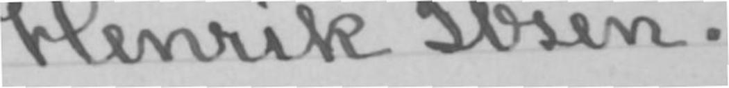Henrik Ibsen.
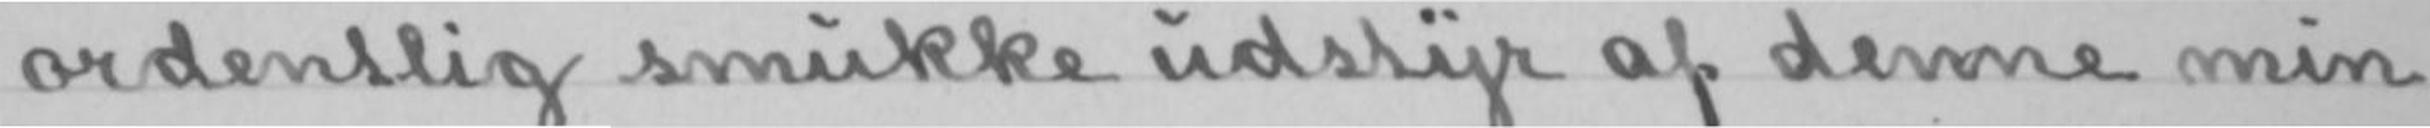ordentlig smukke udstyr af denne min
